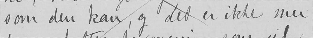som den kan, og det er ikke mer
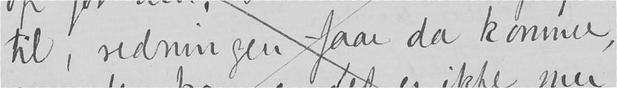til, redningen faar da komme,
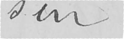sin
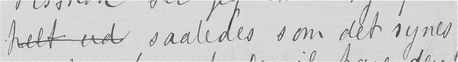helt ud saaledes som det synes
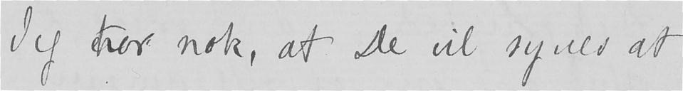Jeg tror nok, at De vil synes at
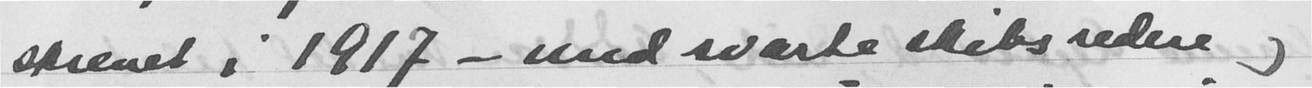skrevet i 1917 - med svarte skibsredere og
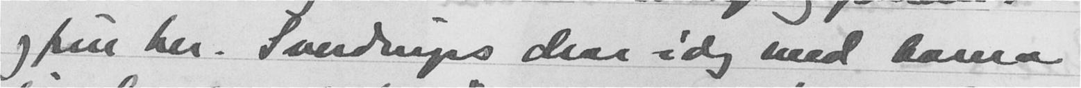og fru her. Sverdrups drar i dag med barna
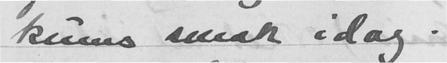kums smak idag.
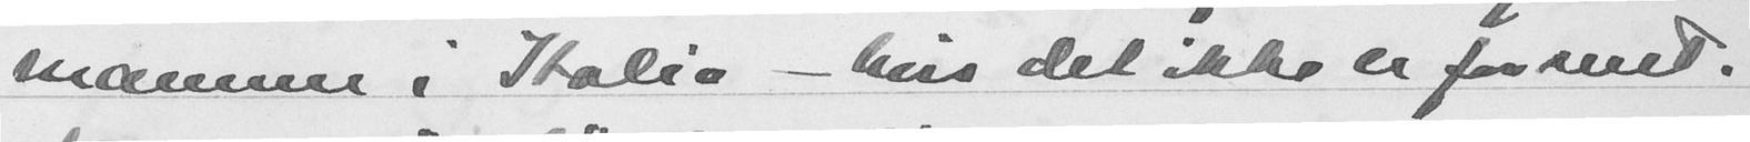mannen i Italia - hvis det ikke er forsent.
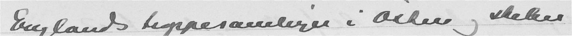Englands troppesamlinger i Østen og skiller
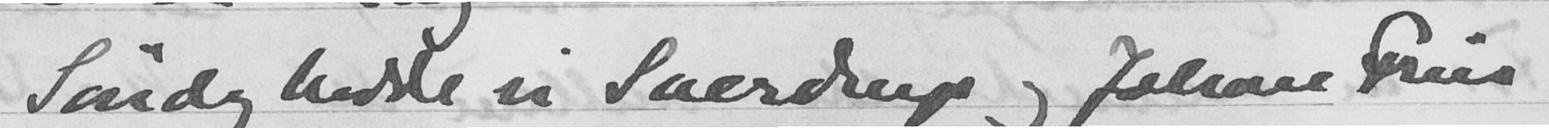Søndag hadde vi Sverdrup og Johan Friis
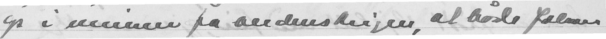op i minnene fra verdenskrigen, at både Johan
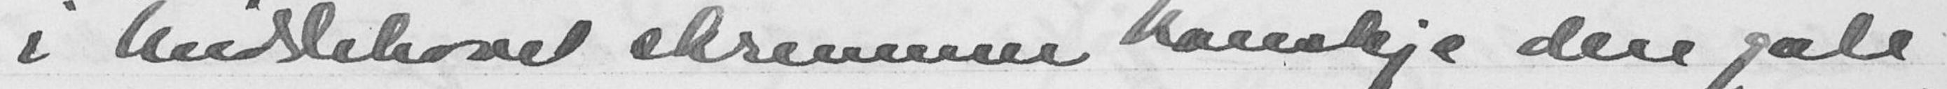i Middelhavet skremmer kanskje den gale
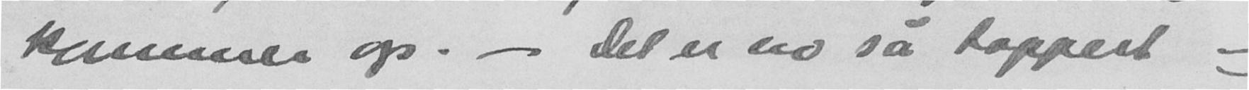kommer op. - Det er en så tappert -
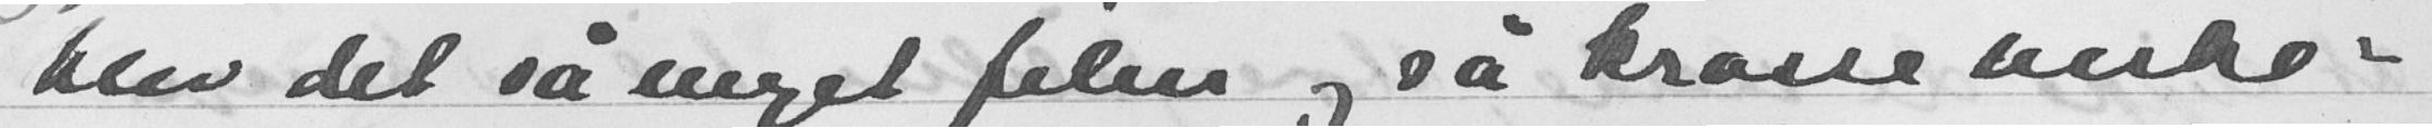blev det så meget film og så krasse virke-
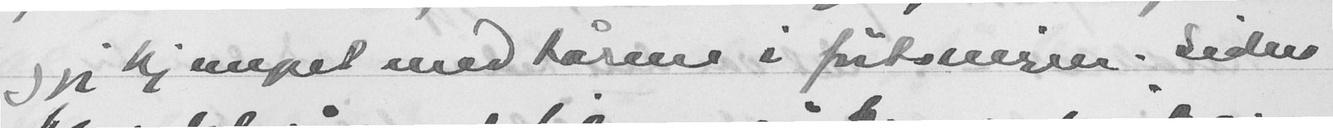og jeg kjempet med tårene i førstningen. Siden
